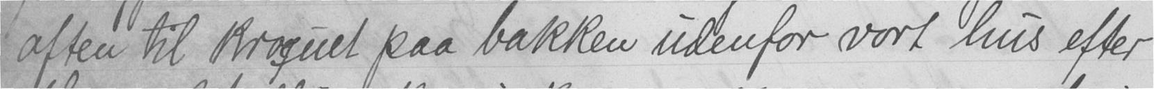aften til kroquet paa bakken udenfor vort hus efter
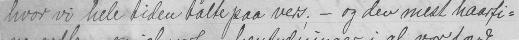hvor vi hele tiden talte paa vers; - og den mest haarfi-
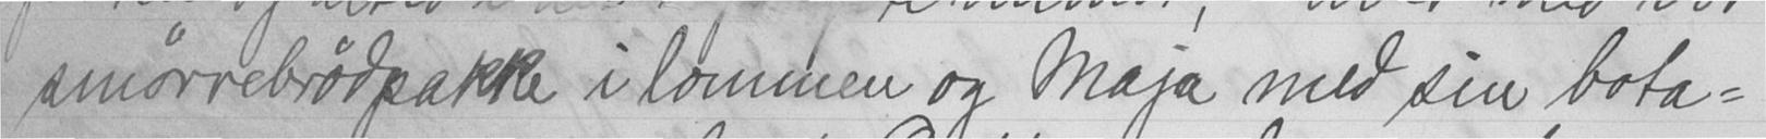smørrebrødpakke i lommen og Maja med sin bota-
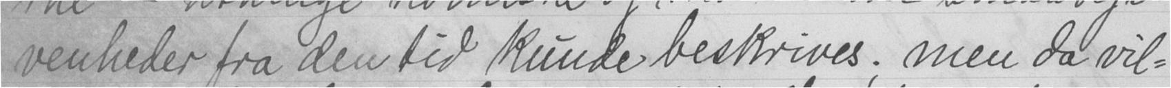venheder fra den tid kunde beskrives; men da vil-
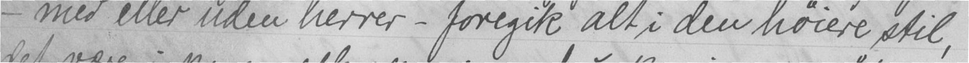- med eller uden herrer - foregik alt i den høiere stil,
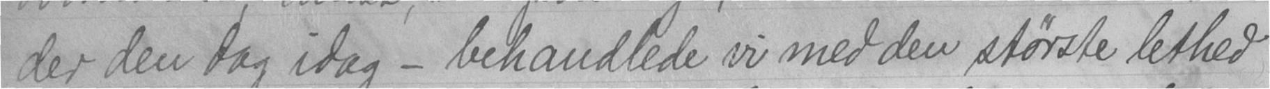der den dag idag - behandlede vi med den største lethed
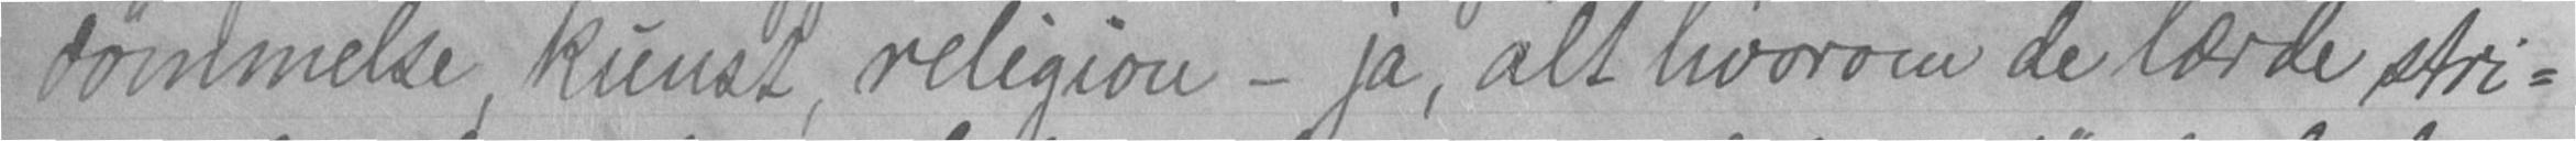dømmelse, kunst, religion - ja, alt hvorom de lærde stri-
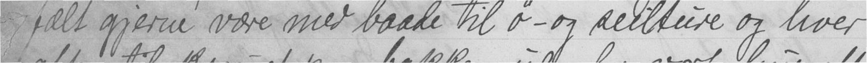fælt gjerne være med baade til ø- og seilture og hver
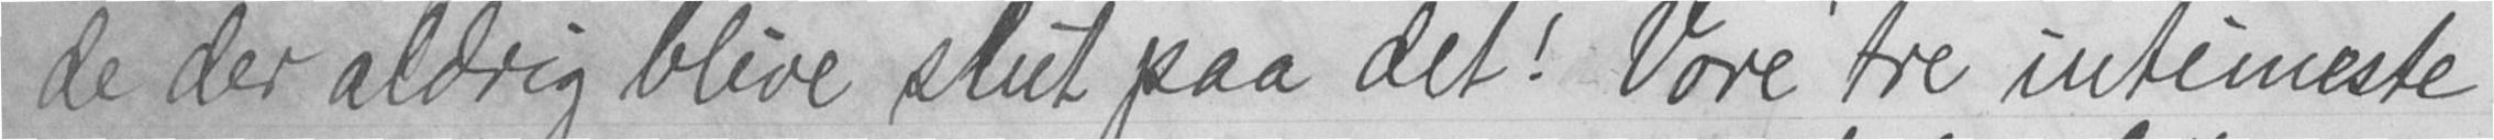de der aldrig blive slut paa det! Vore tre intimeste
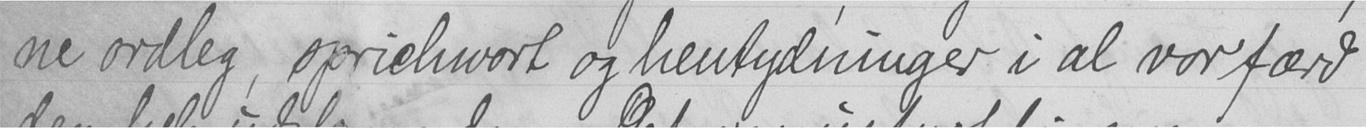ne ordleg, sprichvort og hentydninger i al vor færd
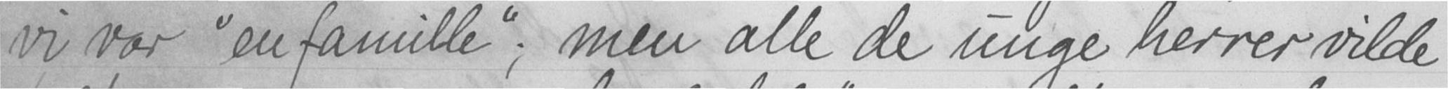vi var "en famille"; men alle de unge herrer vilde
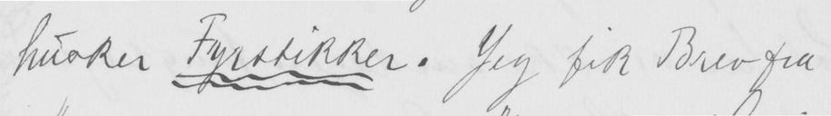husker Fyrstikker. Jeg fik Brev fra
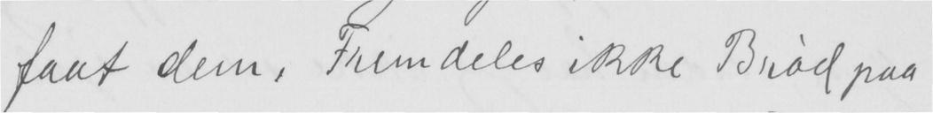faat dem. Fremdeles ikke Brød paa
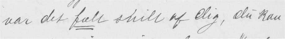var det fælt snilt af dig, du kan
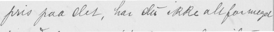pris paa det, har du ikke alt for meget
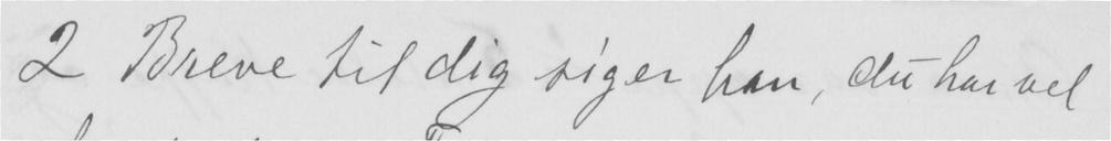2 Breve til dig siger han, du har vel
